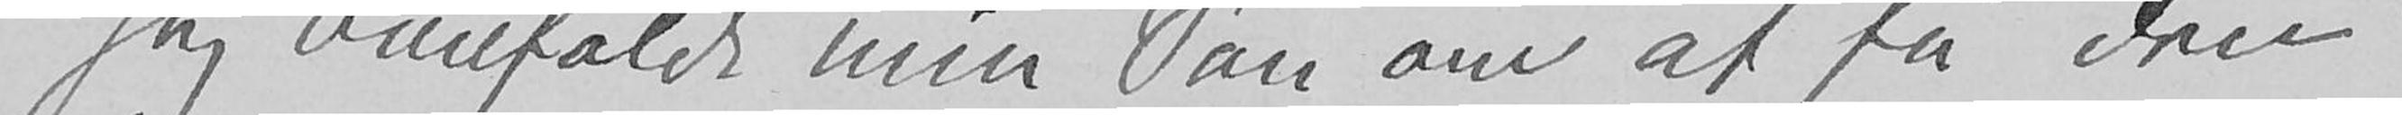Jeg bønfaldt min Søn om at få den
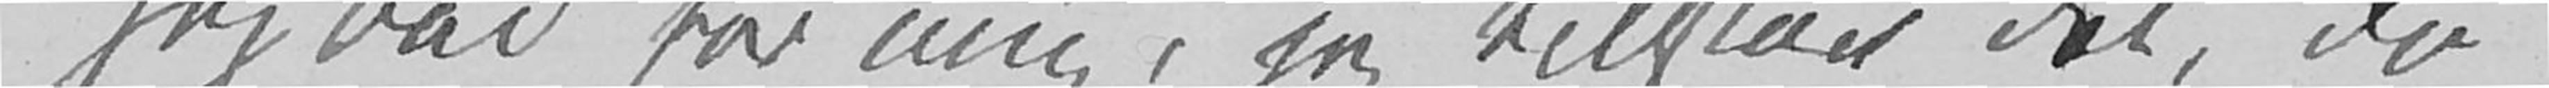Jeg bad for mig, jeg tilstår det, da
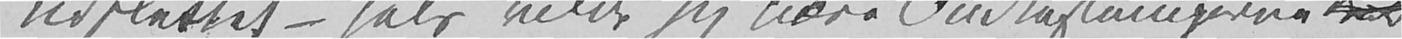udslettet – selv vilde jeg bære Omkostningerne der
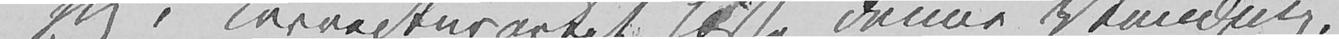jeg i Korrecturarket læste denne Vending.
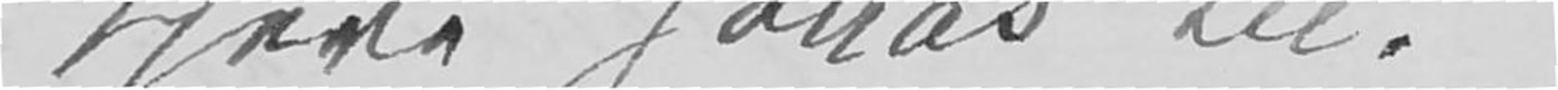Kjere Jonas Lie!
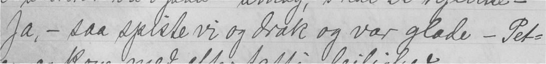Ja, - saa spiste vi og drak og var glade - Pet-
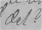det?
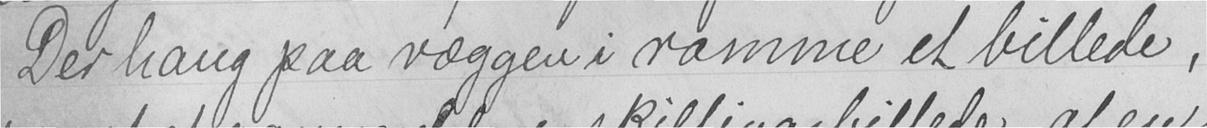Der hang paa væggen i ramme et billede,
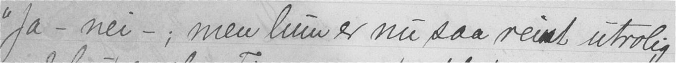"Ja - nei - ; men hun er nu saa reint utrolig
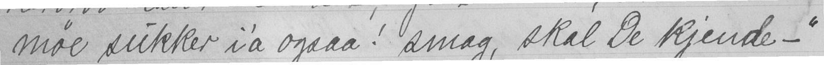møe sukker i'a ogsaa! smag, skal De kjende - "
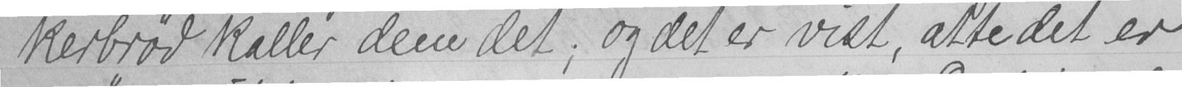kerbrød kaller dem det; og det er vist, atte det er
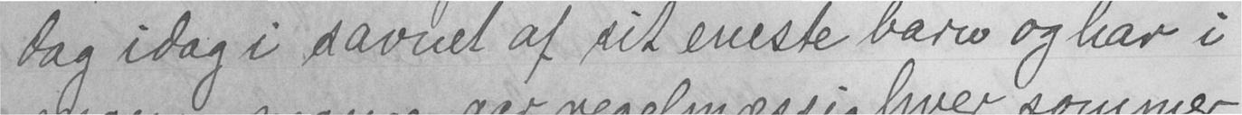dag idag i savnet af sit eneste barn og har i
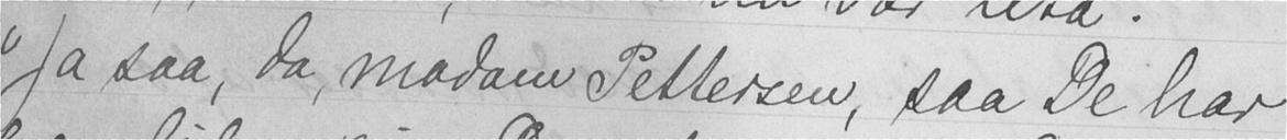"Ja saa, da, madam Pettersen, saa De har
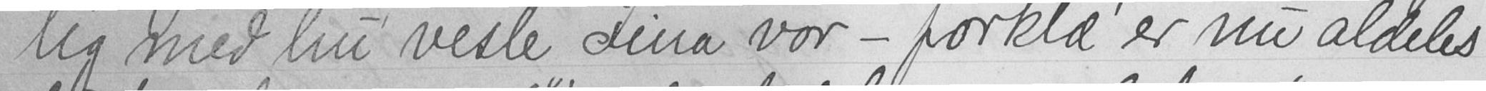lig med hu vesle Tina vor - forklæ' er nu aldeles
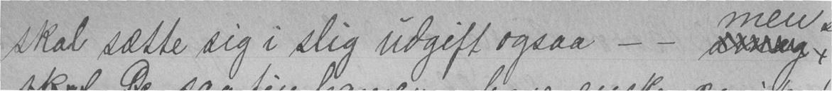skal sætte sig i slig udgift ogsaa - - ueng,
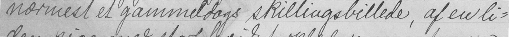nærmest et gammeldags skillingsbillede, af en li-
